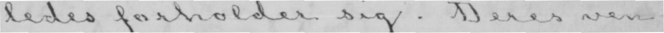ledes forholder sig. Deres ven-
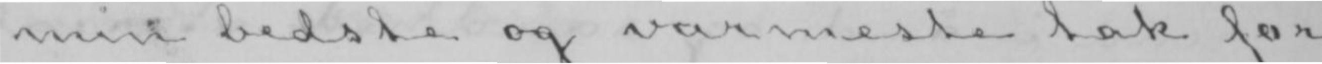min bedste og varmeste tak for
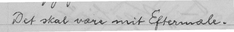Det skal være mit Eftermæle.
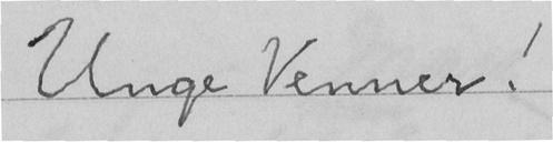Unge Venner!
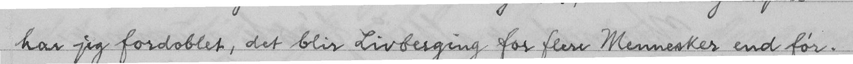har jeg fordoblet, det blir Livberging for flere Mennesker end før.
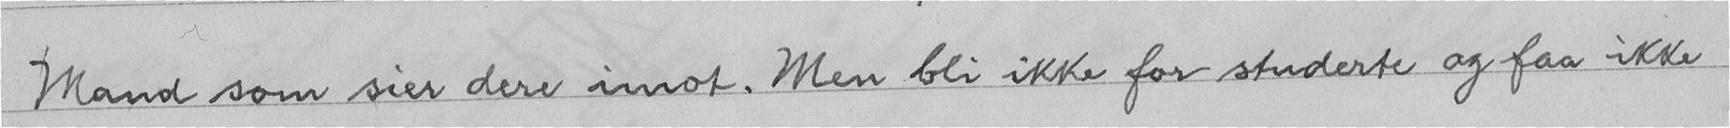Mand som sier dere imot. Men bli ikke for studerte og faa ikke
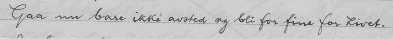Gaa nu bare ikke avsted og bli for fine for Livet.
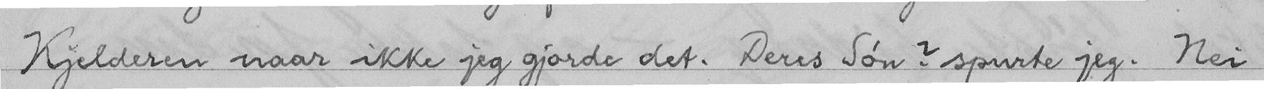Kjelderen naar ikke jeg gjorde det. Deres Søn? spurte jeg. Nei
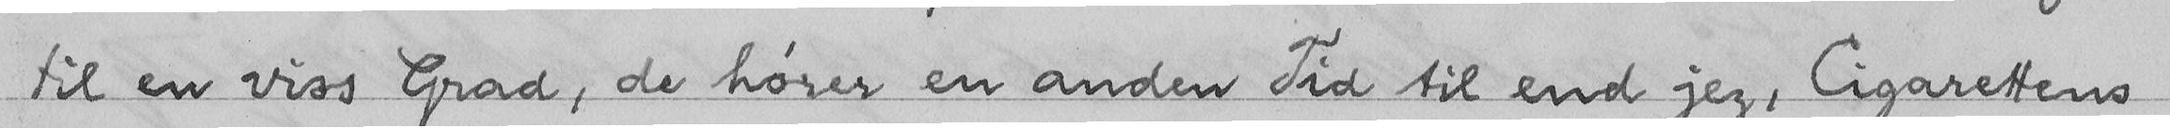til en viss Grad, de hører en anden Tid til end jeg, Cigarettens
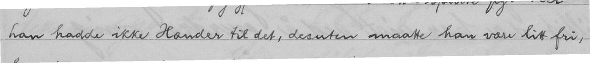han hadde ikke Hænder til det, desuten maatte han være litt fri,
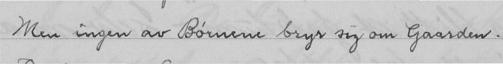Men ingen av Børnene bryr sig om Gaarden.
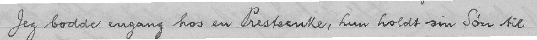Jeg bodde engang hos en Presteenke, hun holdt sin Søn til
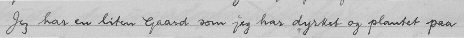Jeg har en liten Gaard som jeg har dyrket og plantet paa
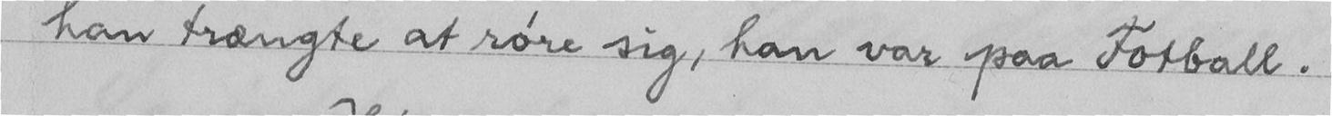han trængte at røre sig, han var paa Fotball.
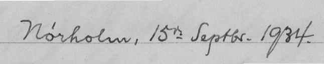Nørholm, 15de Septbr. 1934.
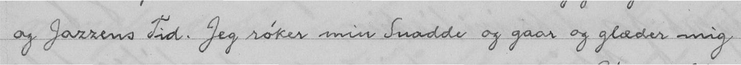og Jazzens Tid. Jeg røker min Snadde og gaar og glæder mig
